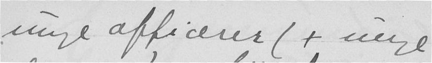unge officerer (+ unge
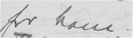for ham.
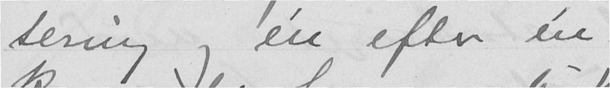tering og én efter én
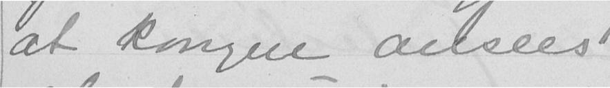at kongen ansees
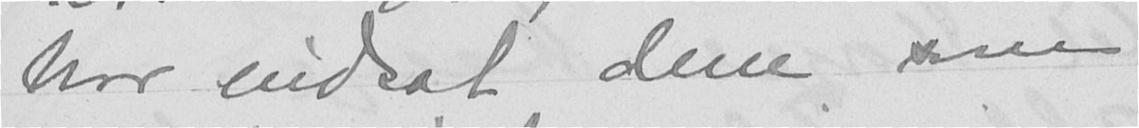har indsat dem som
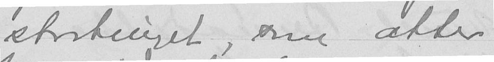stortinget, som atter
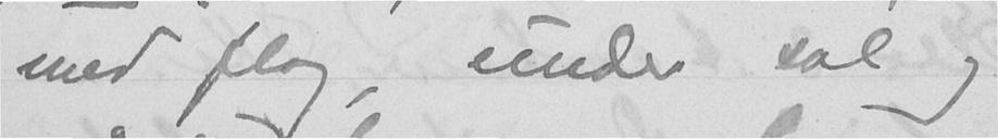med flag, under sol og
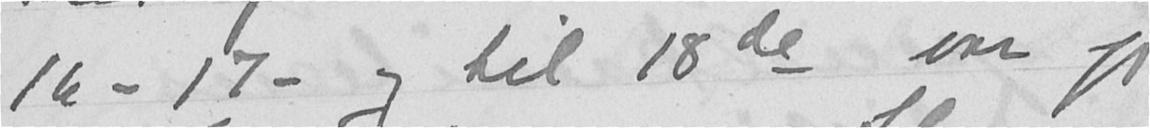16-17- og til 18de var jeg
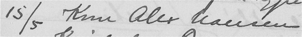15/5 Kom Alex Nansen
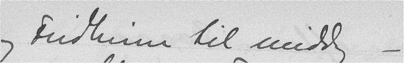og Fridheim til middag -
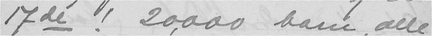17de! 20,000 barn, alle
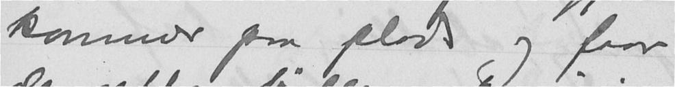kommer paa plads og faar
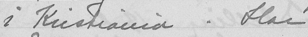i Kristiania. Har
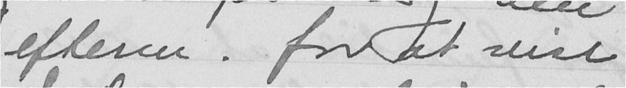efterm. for at reise
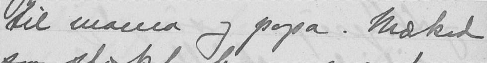til mama og papa. Mærked
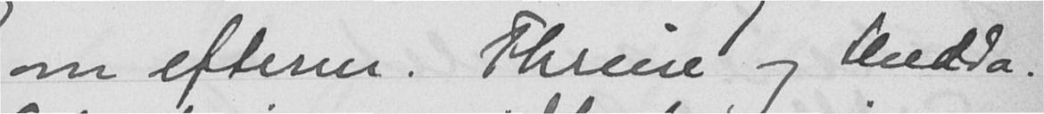om efterm. Thrine og Hulda.
